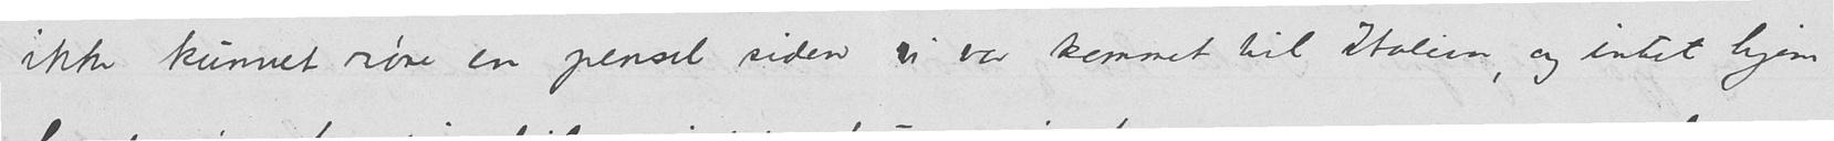ikke kunnet røre en pensel siden vi var kommet til Italien og intet hjem
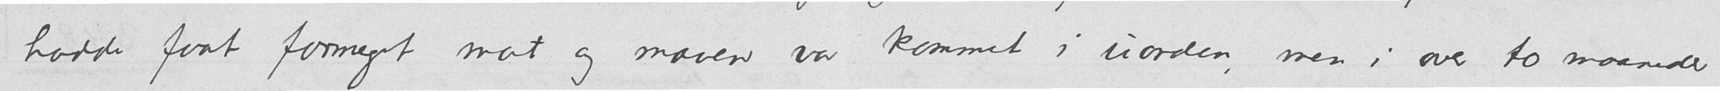havde faat formeget mat og maven var kommet i uorden, men i over to maaneder
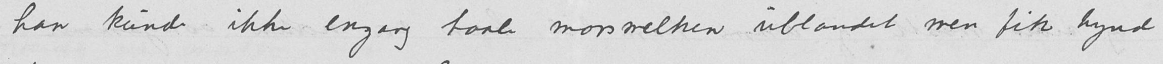han kunde ikke engang taale morsmelken ublandet men fik tynd
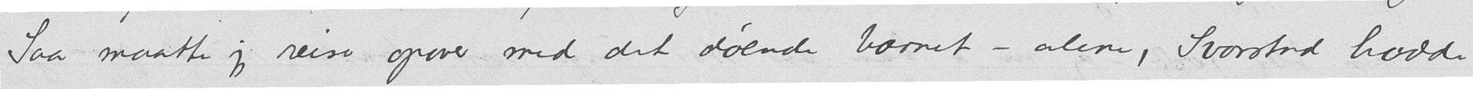Saa maatte jeg reise opover med det døende barnet - alene, Svarstad hadde
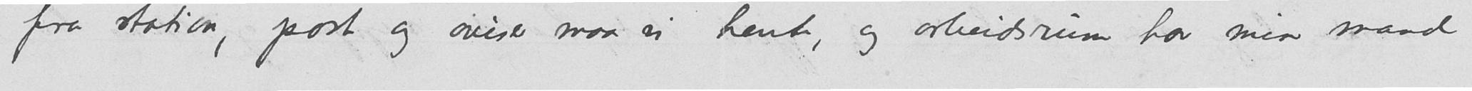fra station, post og aviser maa vi hente, og arbeidsrum har min mand
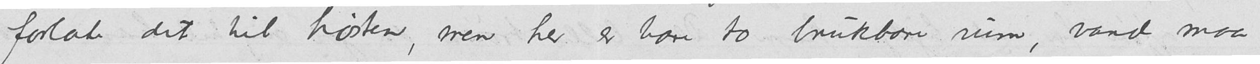forlate det til høsten, men her er bare to brukbare rom, vand maa
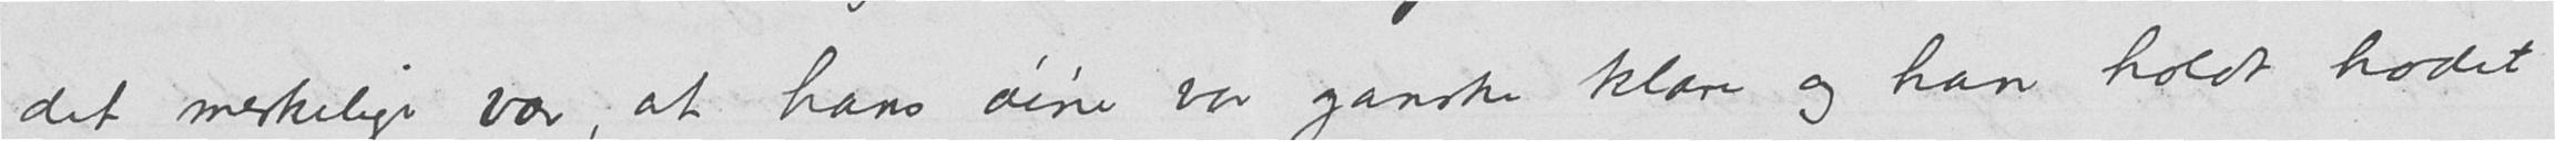det merkelige var, at hans øine var ganske klare og han holdt hodet
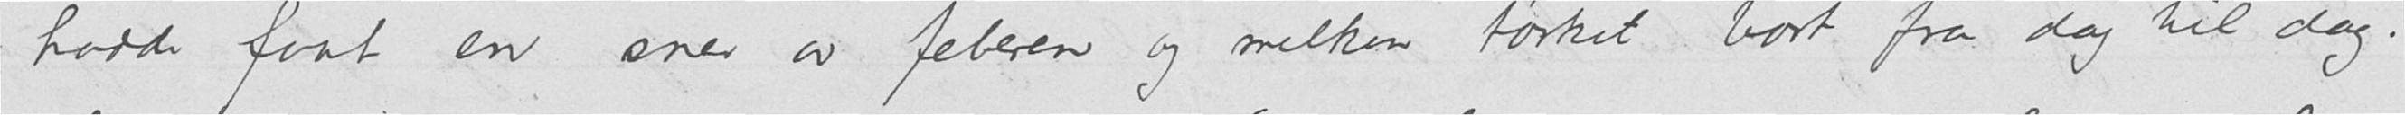havde faat en snev av feberen og melken tørket bort fra dag til dag.
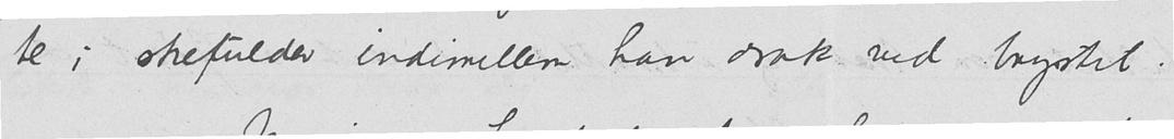te i skefulder indimellem han drak ved brystet.
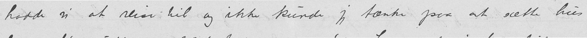hadde vi at reise til og ikke kunde jeg tænke paa at sætte hus
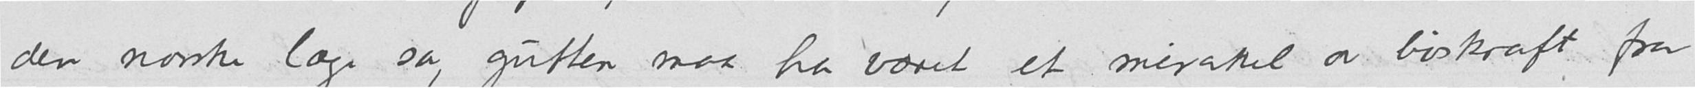den norske lage sa, gutten maa ha været et mirakel av livskraft fra
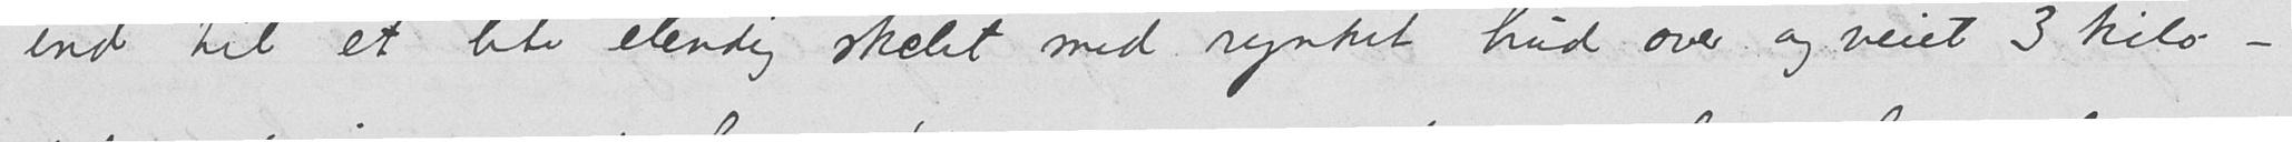ind til et lite elendig skelet med rynket hud over og veiet 3 kilo -
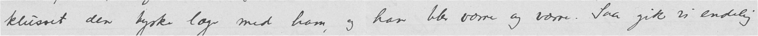klusset den tyske læge med ham, og han blev værre og værre. Saa gik vi endelig
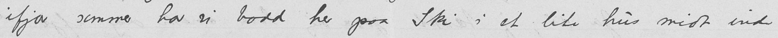ifjor sommer har vi bodd her paa Ski i et lite hus midt ind
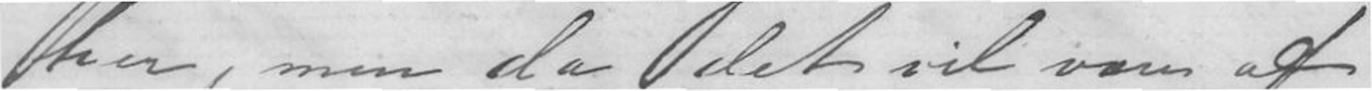her, men da det vil være af
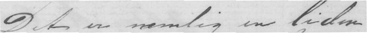-Det er nemlig en liden
